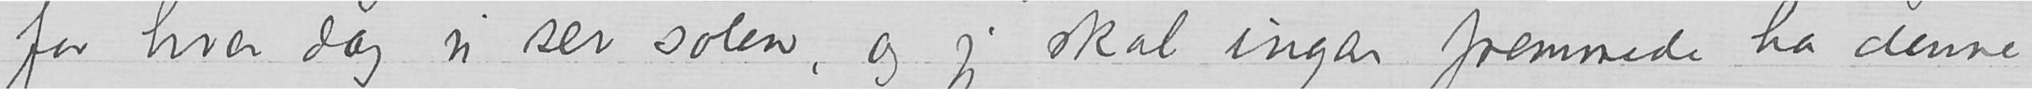for hver dag vi ser solen, og jeg skal ingen fremmede ha denne
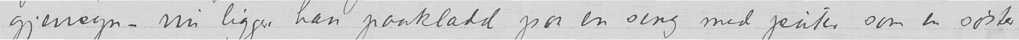gjensyn. Nu ligger han paaklædd paa en seng med puter som en søster
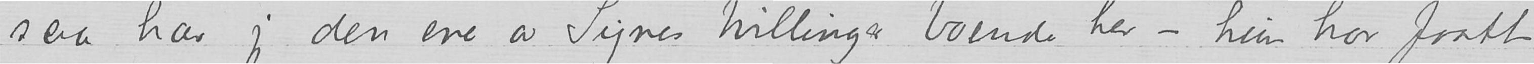saa har jeg den ene av Signes tvillinger boende her – hun har faatt
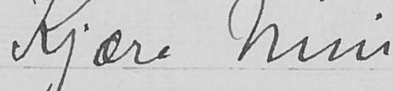Kjære Nini
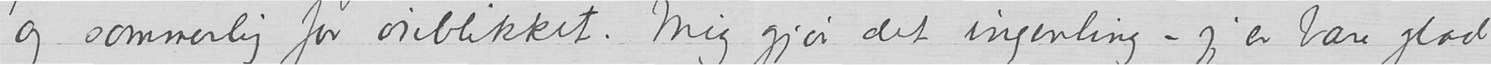og sommerlig for øieblikket. Mig gjør det ingenting – jeg er bare glad
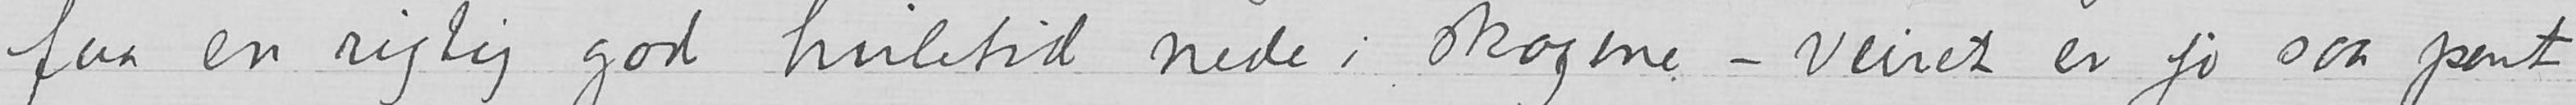faa en rigtig god hviletid nede i skogene – veiret er jo saa pent
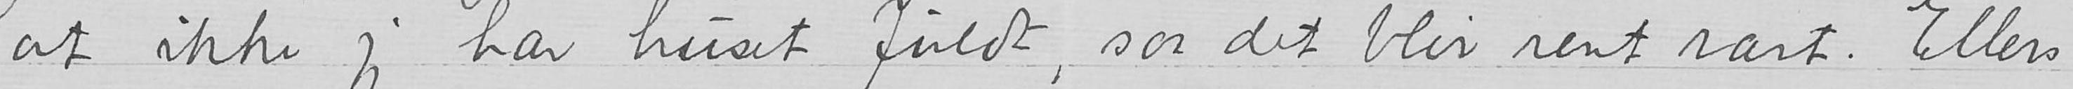at ikke jeg har huset fuldt, saa det blir rent rart. Ellers
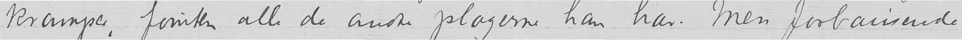kramper, foruten alle de andre plagerne han har. Men forbausende
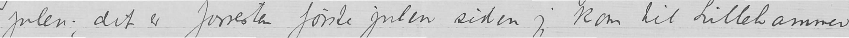julen, det er forresten første julen siden jeg kom til Lillehammer
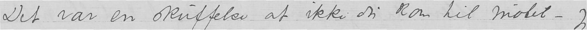Det var en skuffelse at ikke du kom til møtet – jeg
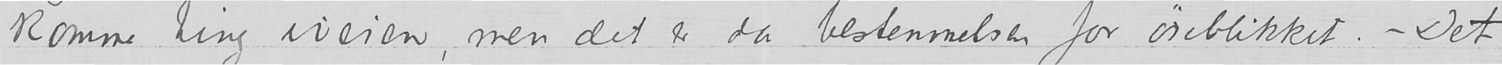komme ting iveien, men det er da bestemmelsen for øieblikket. – Det
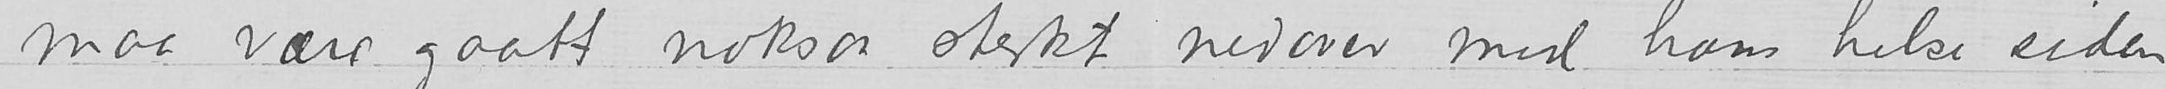maa være gaatt nokså sterkt nedover med hans helse siden
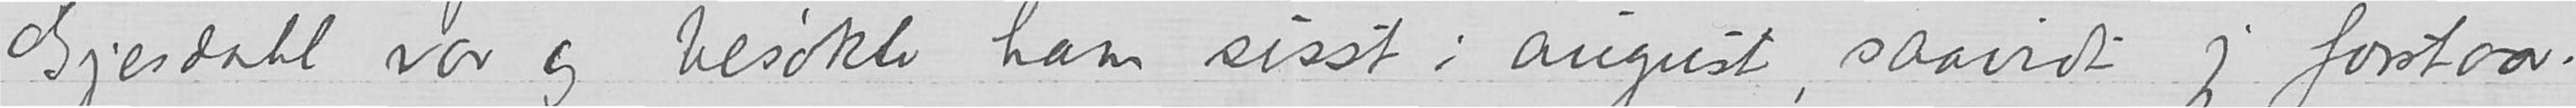Gjesdahl var og besøkte ham sisst i august, saavidt jeg forstaar.
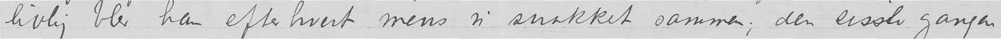livlig blev han efterhvert mens vi snakket sammen; den sisste gangen
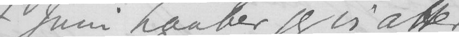I Juni haaber jeg vi atter
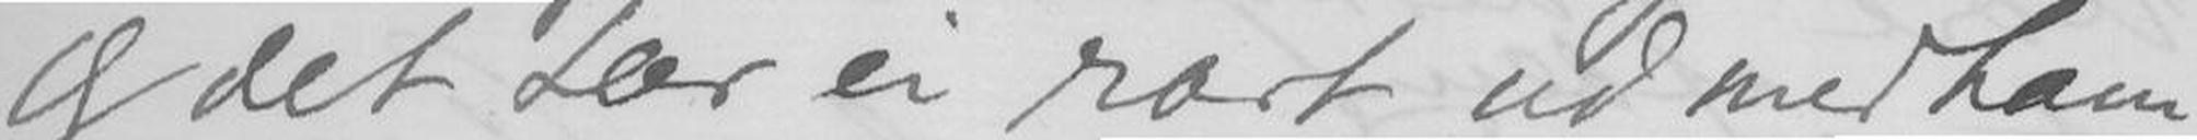og det ser ei rart med ham.
